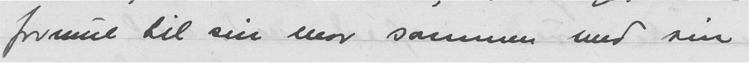formue til sin mor sammen med sin
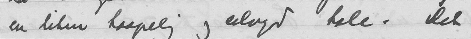en liten taapelig og selvyd tale. Det
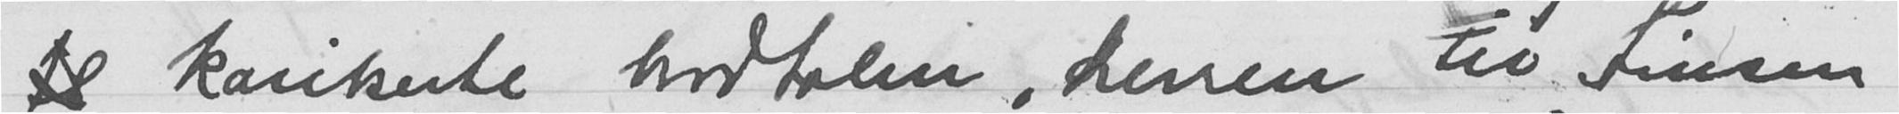fl karikerte bordtalen, herren til Sinsen
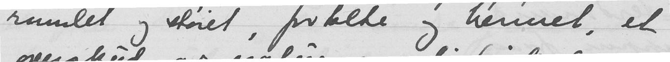rumlet og støret, fortalte og hermet, et
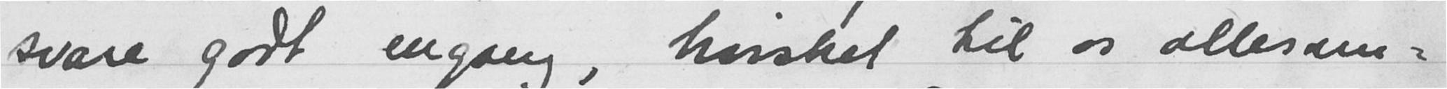svare godt engang, hvisket til os allesam-
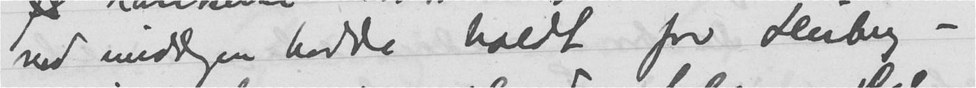ved middagen hadde holdt for Heiberg -
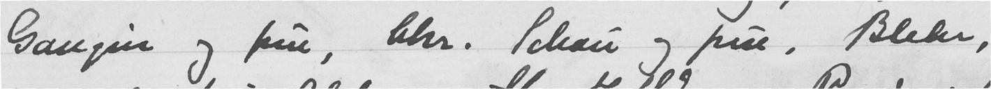Gaugin og frue, Chr. Schou og frue, Blehr,
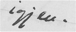igjen.
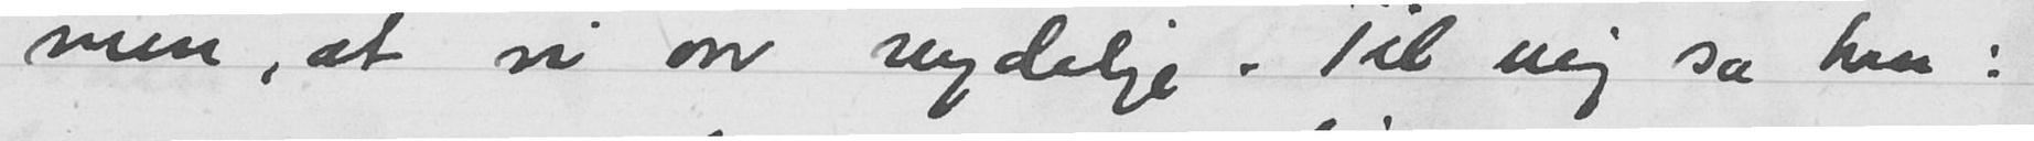men, at vi var nydelige. Til mig sa han:
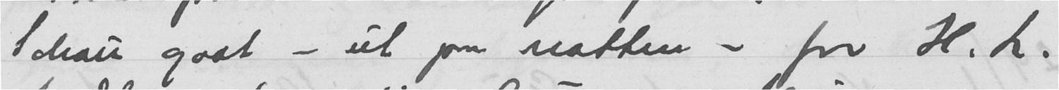Schou gaat - ut paa natten - for H. L.
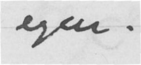egen.
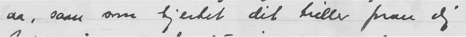aa, saan som hjertet dit triller foran dig
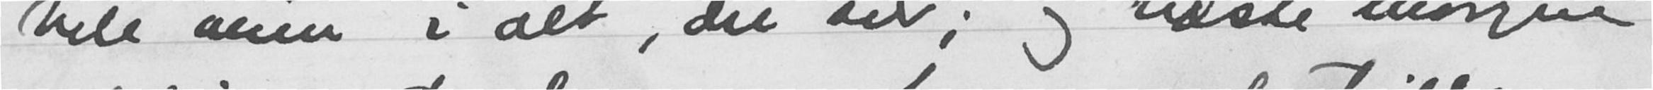hele veien i alt, du sier; og næste morgen
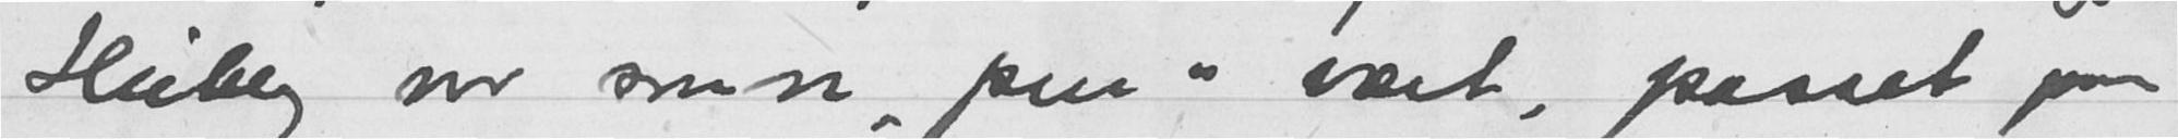Heiberg var saan "pen" vært, passet paa
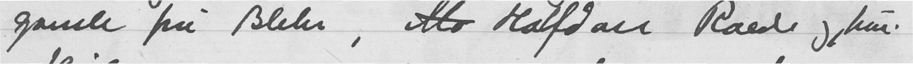gamle fru Blehr, Mo Halfdan Raabe og frue.
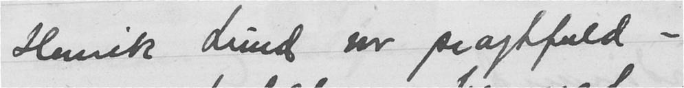Henrik Lund var pragtfuld -
